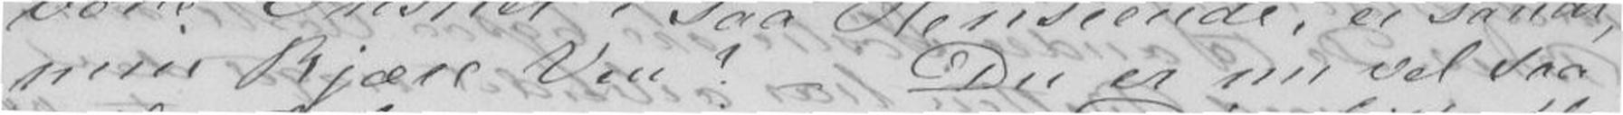min kjære Ven? - Du er nu vel saa
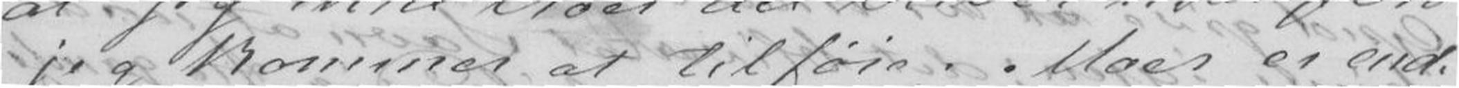jeg kommer at tilføie. Moer er end-
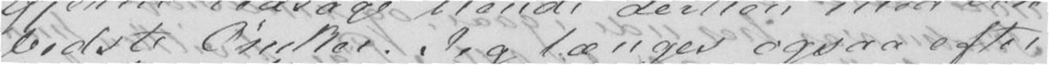bedste Ønsker. Jeg længes ogsaa efter
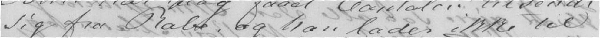sig fra Rabe, og han lades ikke til
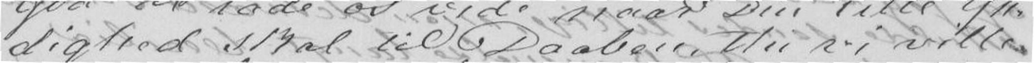dighed skal til Daaben, thi vi ville
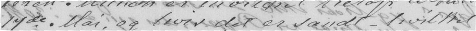17de Mai, og hvis det er sandt- hvilket
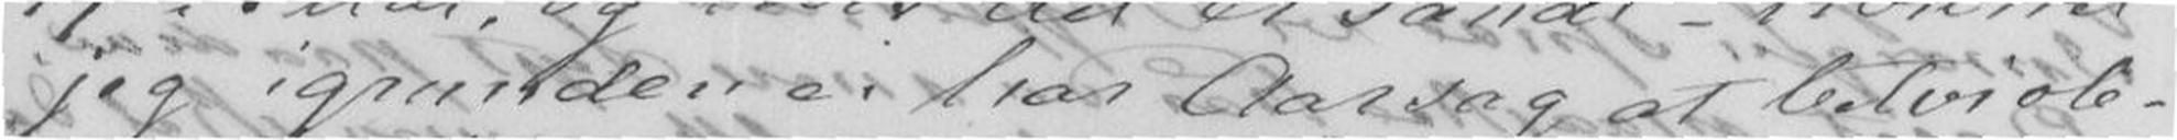jeg igrunden har Aarsag at betvivle
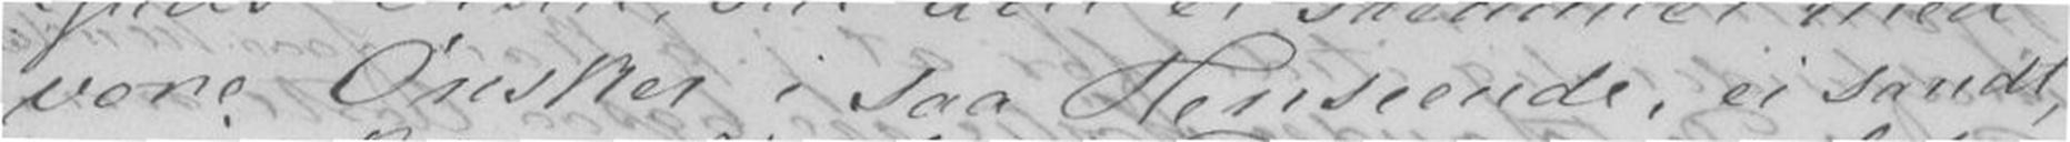vore Ønsker i saa Henseende, ei sandt
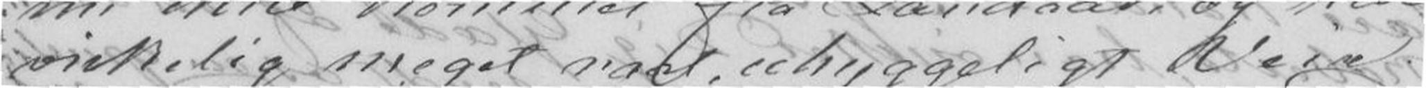virkelig meget raat, uhyggelig Veir.
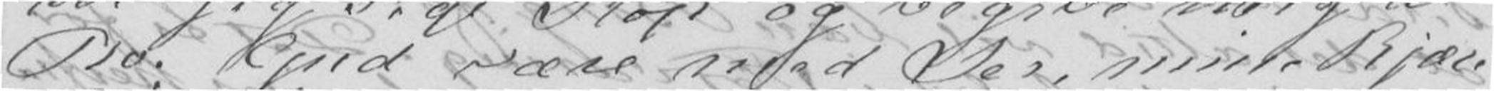Ro. Gud være med Jer, mine kjære
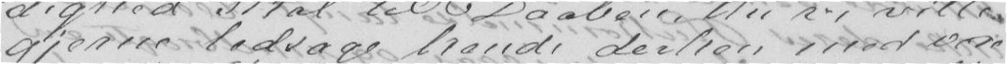gjerne ledsage hende derhen med vore
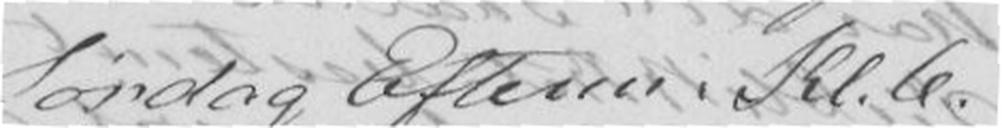Lørdag Efterm. Kl.6.
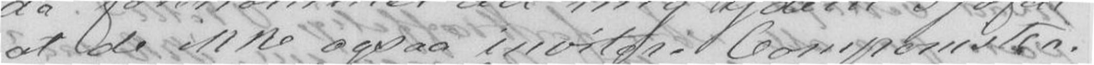at de ikke ogsaa invitere Componisten.
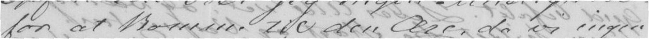for at komme til den Ære, da vi ingen
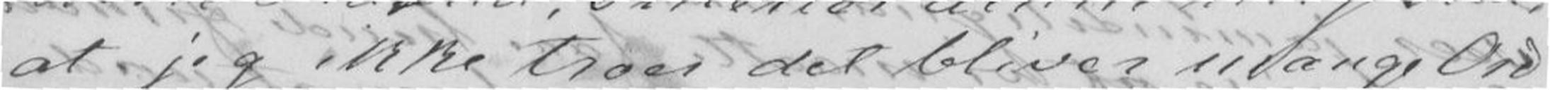at jeg ikke troer det bliver mange Ord
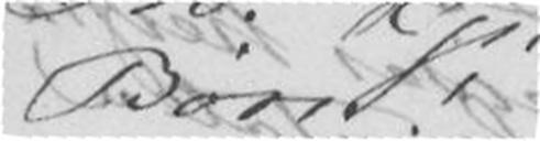Børn
